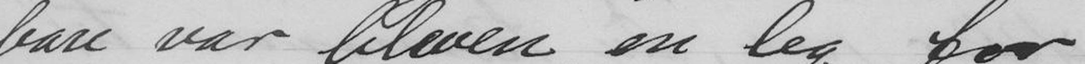bare var bleven en leg for
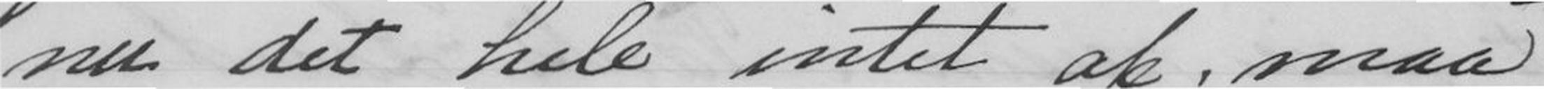nu det hele intet af, maa
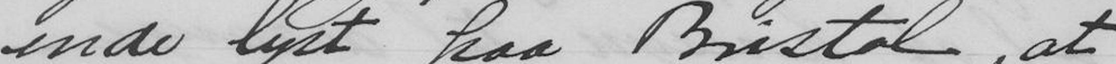ende lyst paa Bristol, at
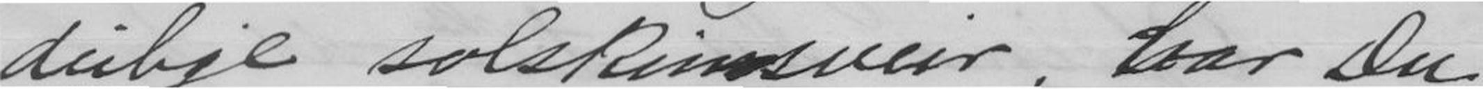deilige solskinsveir, var Du
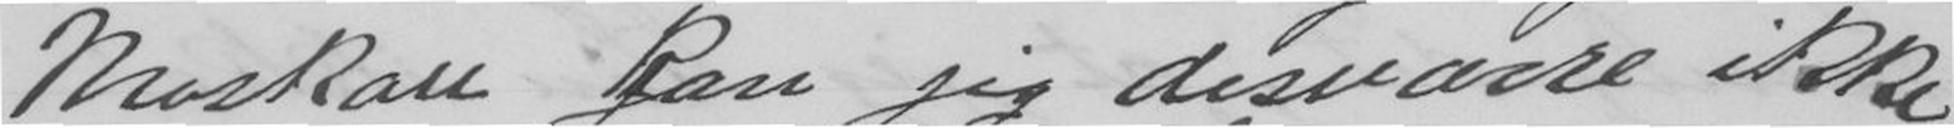Moskau kan jeg desværre ikke
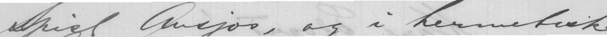spist Ansjos, og i hermetiske
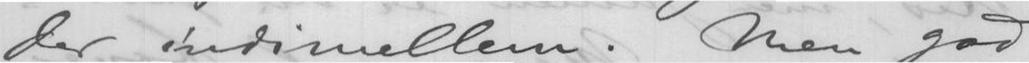der indimellem. Men god
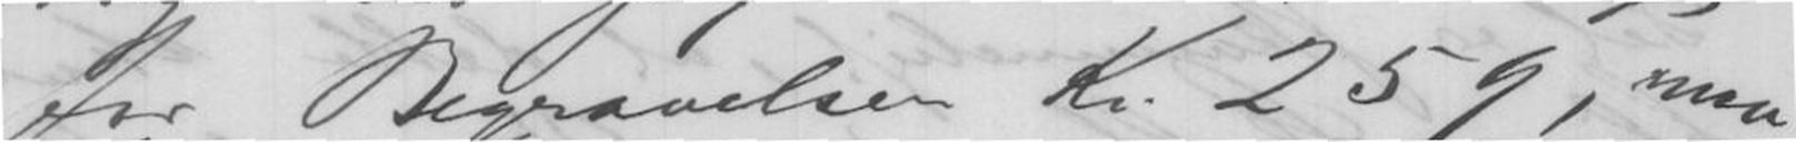for Begravelsen Kr. 259, men
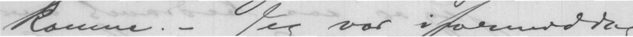komme. - Jeg var iformiddag
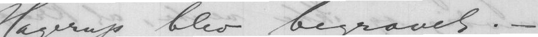Hagerup blev begravet. -
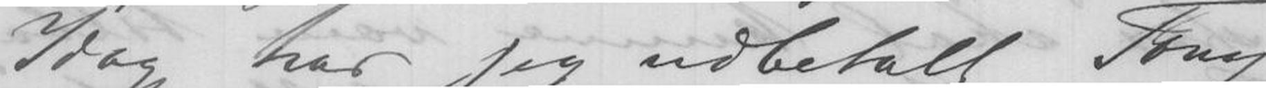Idag har jeg udbetalt Tony
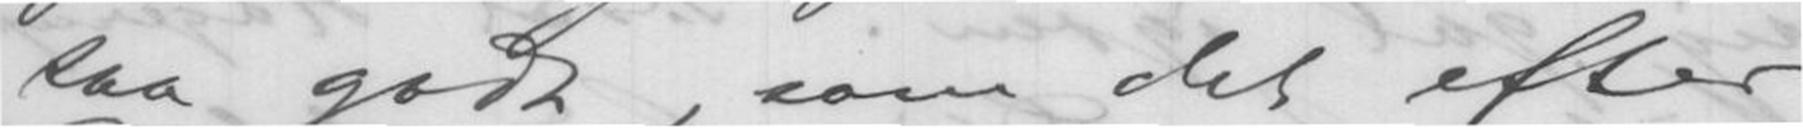saa godt, som det efter
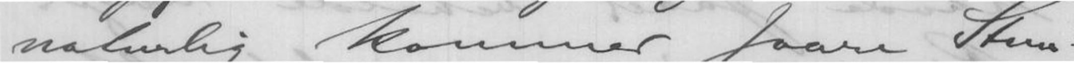naturlig kommer saare Stun-
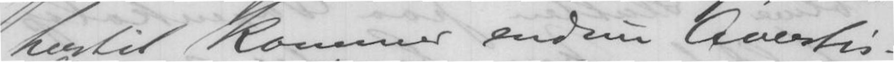hertil kommer endnu Avertis-
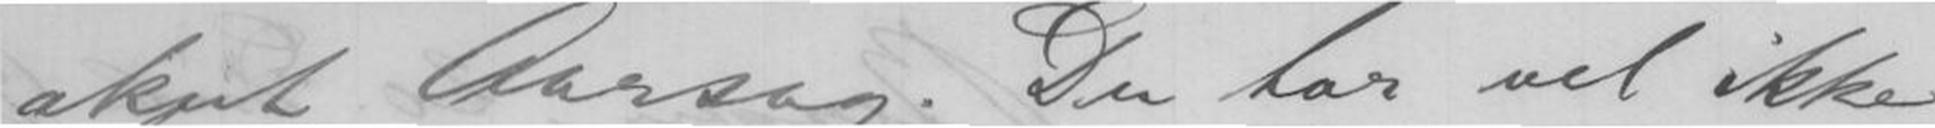akut Aarsag. Du har vel ikke
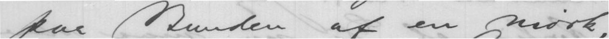paa Bunden af en mørk,
